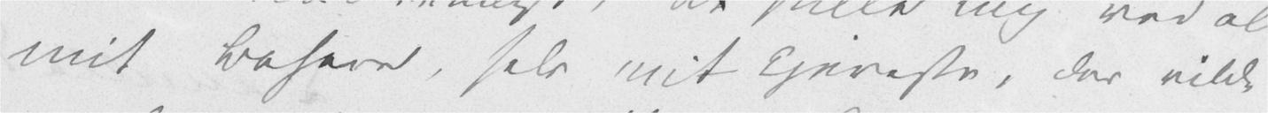mit Bohave, selv mit kjereste, der vilde
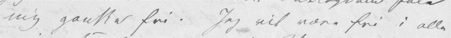mig ganske fri. Jeg vil være fri i alle
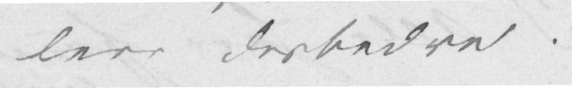leve desbedre.
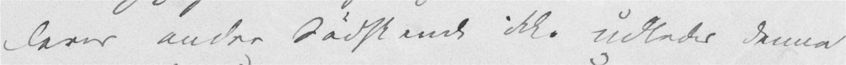Deres andre Sødskende ikke udleder denne
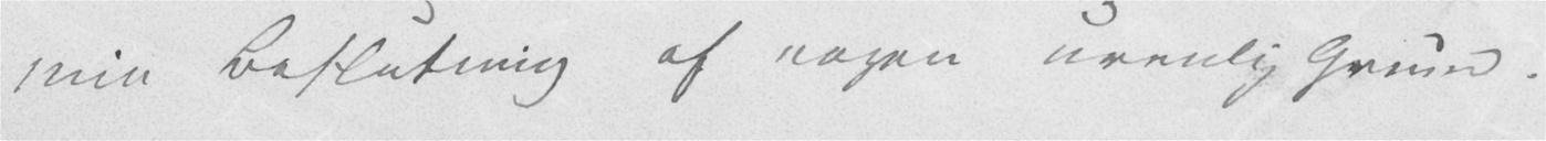min Beslutning af nogen uvenlig Grund.
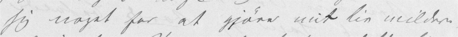sig noget for at gjøre mit Liv mildere,
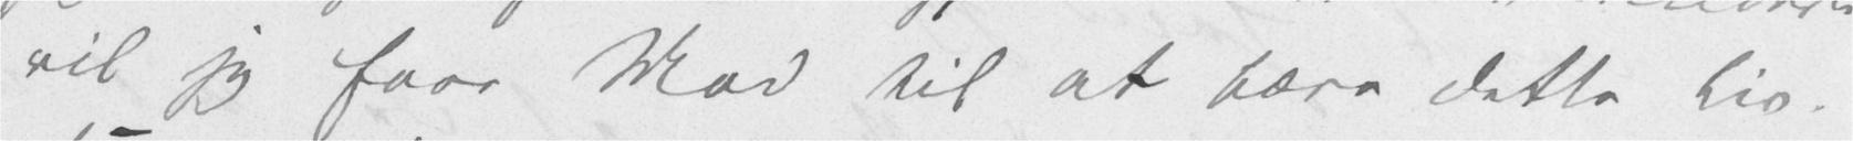vil jeg faae Mod til at bære dette Liv.
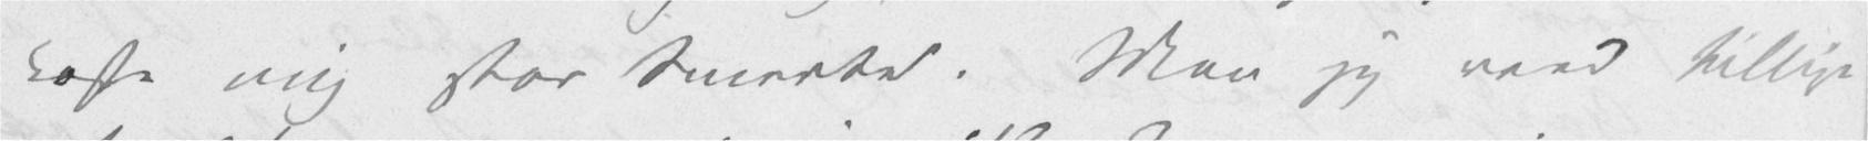koste mig stor Smerte. Men jeg veed tillige
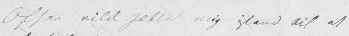Offer vilde sætte mig istand til at
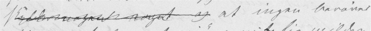skylder nogen noget og at ingen berøver
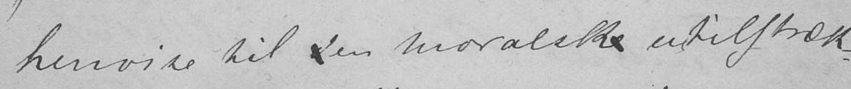henvise til den moralske utilstræk-
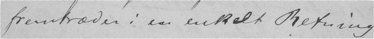fremtræder i en enkelt Retning,
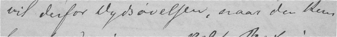vil derfor Dydsøvelsen, naar den kun
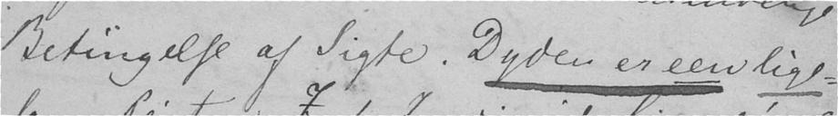Betingelse af Sigte. Dyden er een lige-
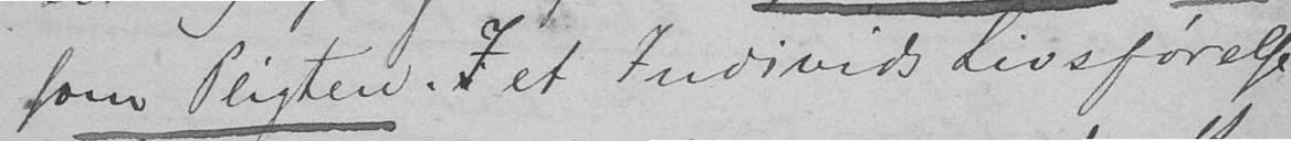som Pligten. I et Individs Livsførelse
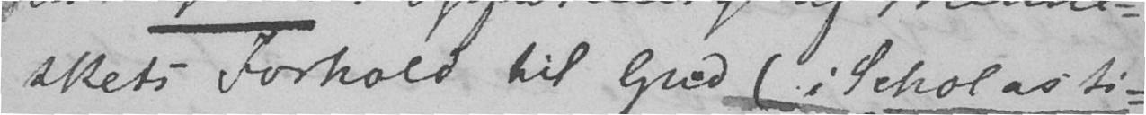skets Forhold til Gud (i Scholasti-
